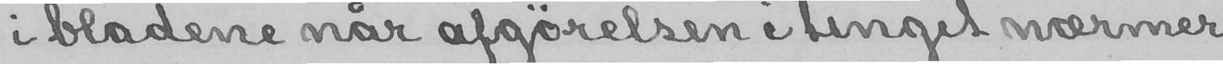i bladene når afgørelsen i tinget nærmer
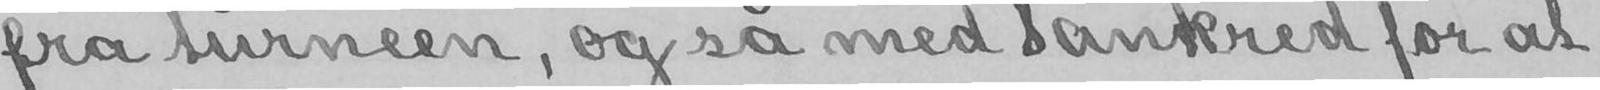fra turnéen, og så med Tankred for at
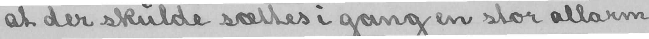at der skulde sættes i gang en stor allarm
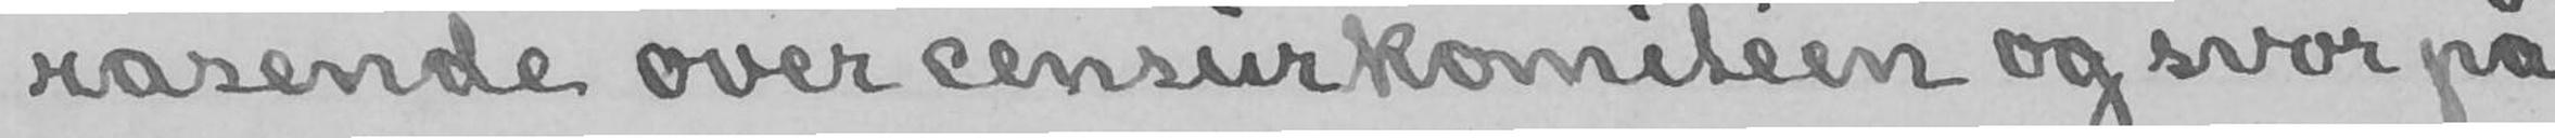rasende over censurkomitéen og svor på
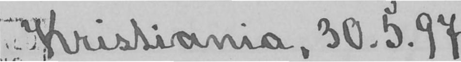Kristiania, 30.5.97.
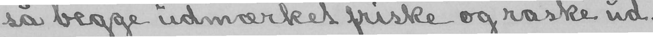så begge udmærket friske og raske ud.
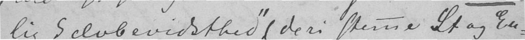lig Selvbevidsthed" (deri stemne St og Eu-
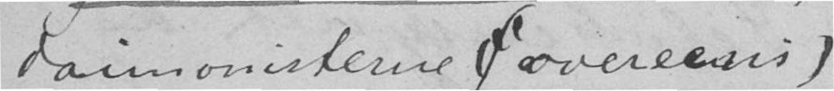daimonisterne overeens)
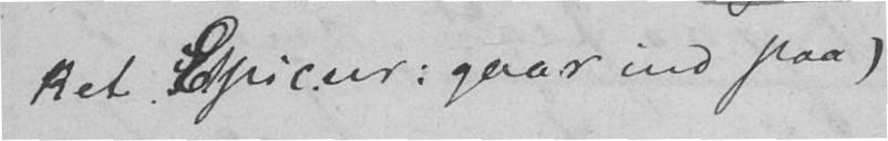ket Epicur: gaar ind paa)
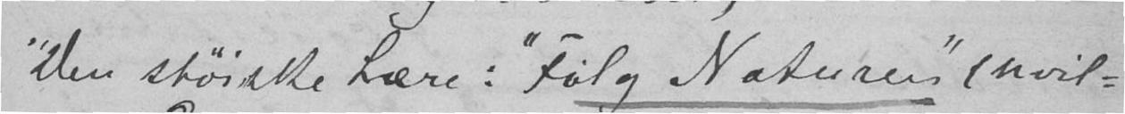Den stoiske Lære: "Følg Naturen (hvil-
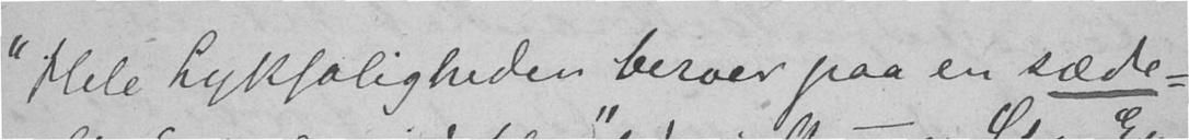"Hele Lyksaligheden beroer paa en sæde-
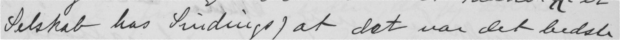Selskab hos Sindings) at det var det bedste
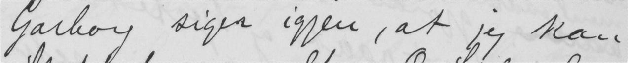Garborg siger igjen, at jeg kan
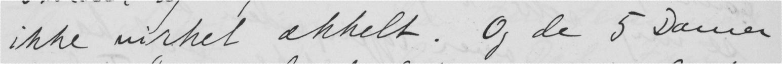ikke virket ækkelt. Og de 5 Damer
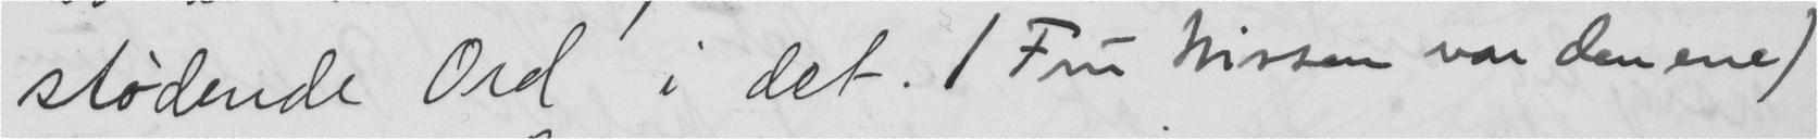stødende Ord i det. ( Fru Nissen var den ene)
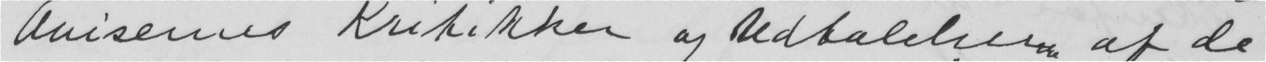avisernes Kritikker og Udtalelser af de
